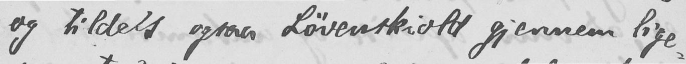og tildels ogsaa Løvenskiold gjennem lige-
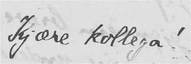Kjære kollega!
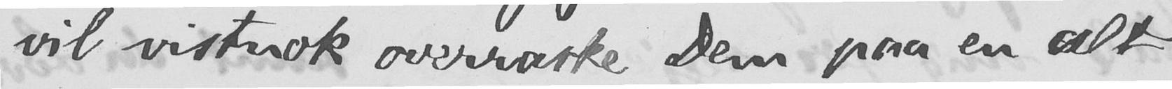vil vistnok overraske Dem paa en alt
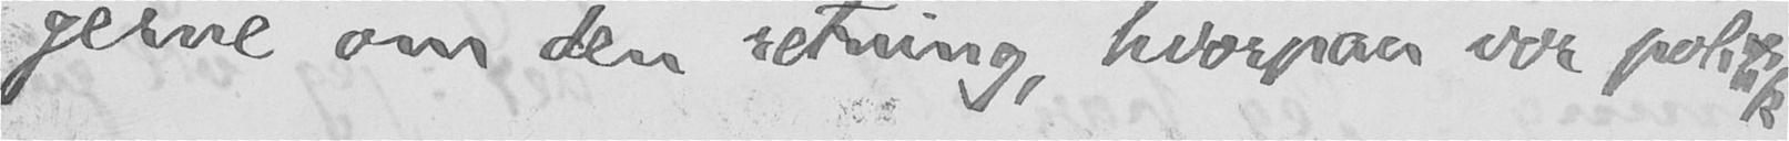gerne om den retning, hvorpaa vor politik
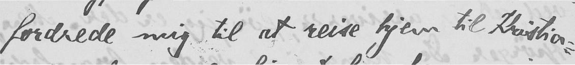fordrede mig til at reise hjem til Kristia-
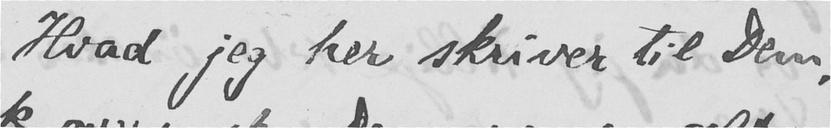Hvad jeg her skriver til Dem,
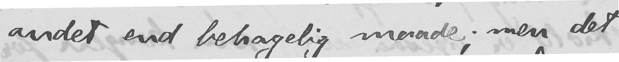andet end behagelig maade; men det
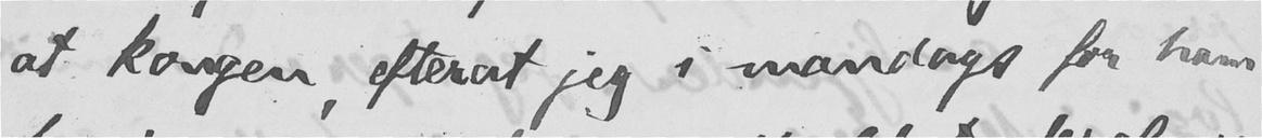at kongen, efterat jeg i mandags for ham
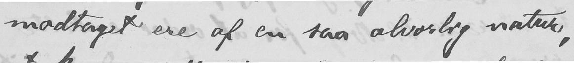modtaget ere af en saa alvorlig natur,
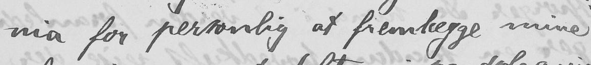nia for personlig at fremlægge mine
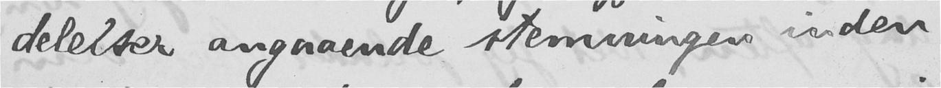delelser angaaende stemningen inden
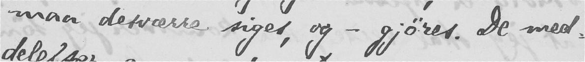maa desværre siges, og - gjøres. De med-
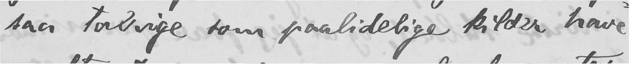saa talrige som paalidelige kilder have
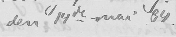den. 14de mai 84.
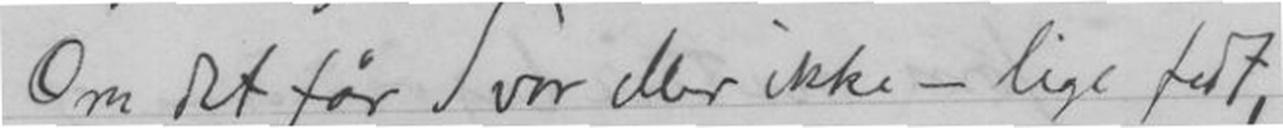Om det får Svar eller ikke - lige fuldt,
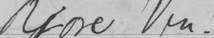Kjære Ven!
